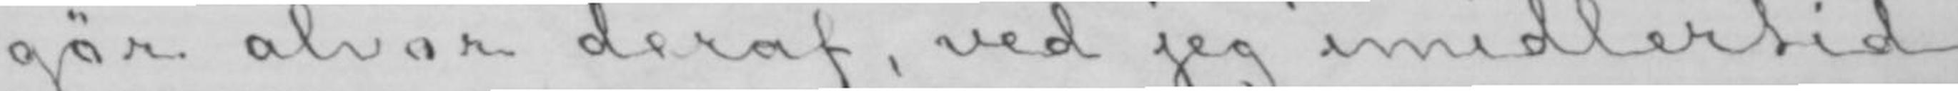gør alvor deraf, ved jeg imidlertid
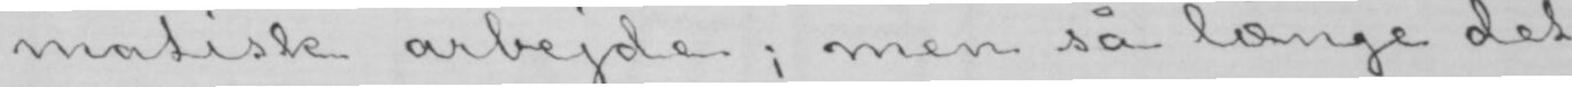matisk arbejde; men så længe det
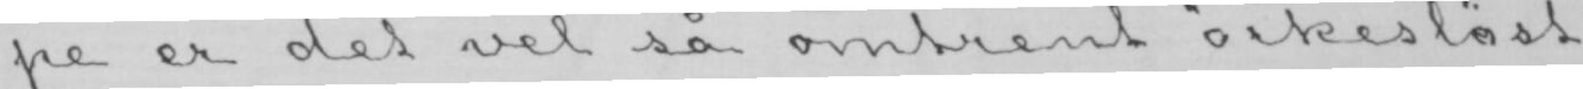pe er det vel så omtrent ørkesløst
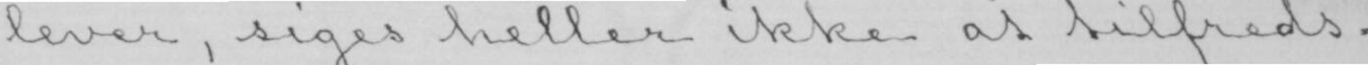lever, siges heller ikke at tilfreds-
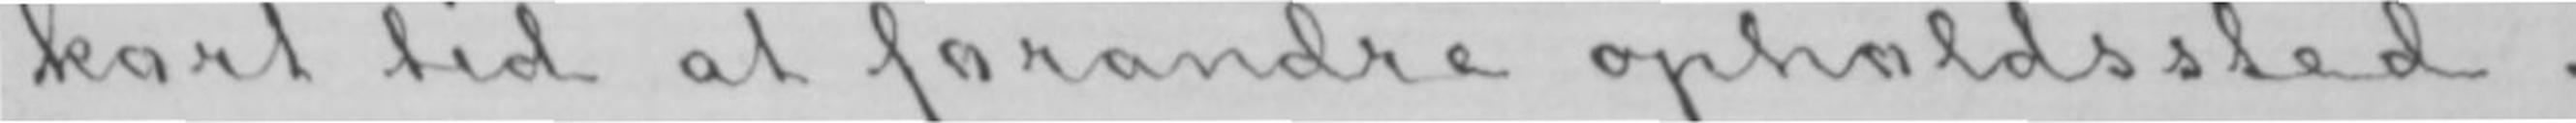kort tid at forandre opholdssted.
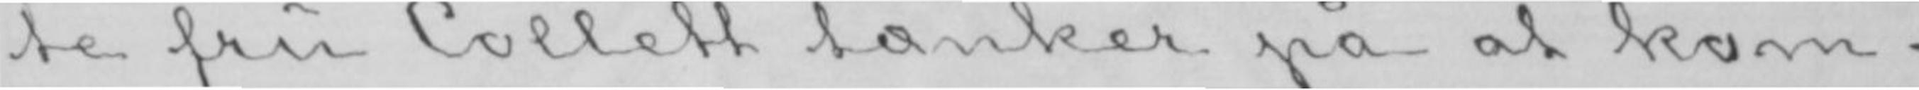te fru Collett tænker på at kom-
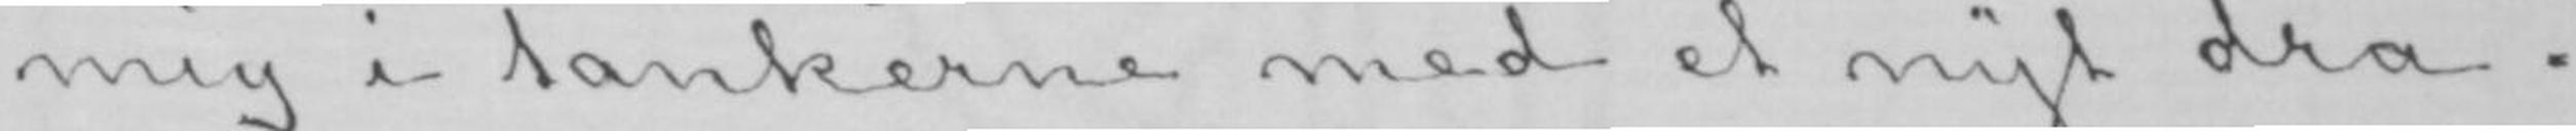mig i tankerne med et nyt dra-
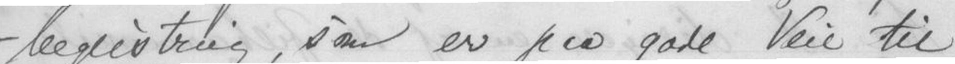-begeistring, som er paa gode Veie til
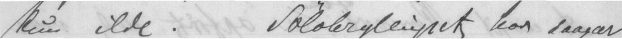kun ilde.Sølvbryllupet kan saagar
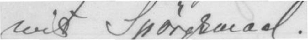mit Spørgsmaal.
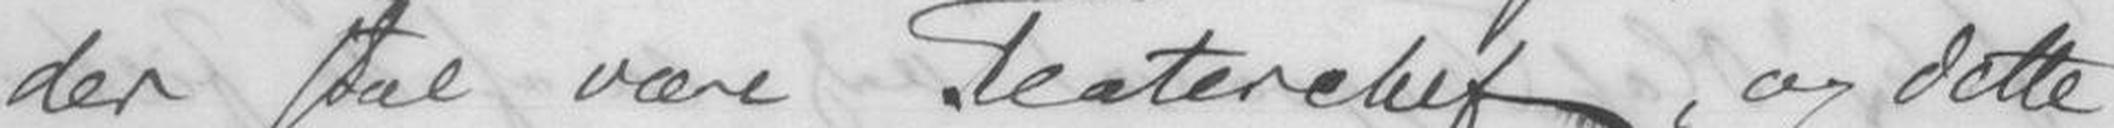der skal være Teaterchef, og dette
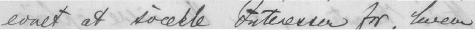at svække Interessen for, hvem
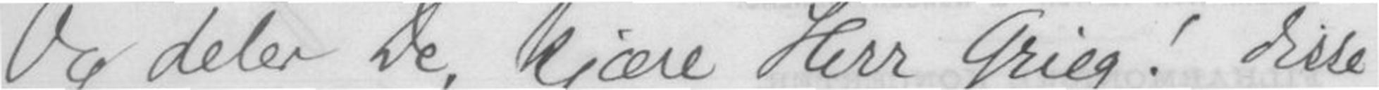Og deler De kjære Herr Grieg ! disse
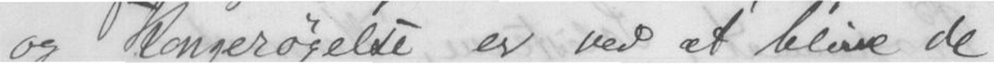og Kongerøgelse er ved at blive de
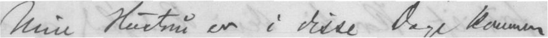Min Hustru er i disse Dage kommen
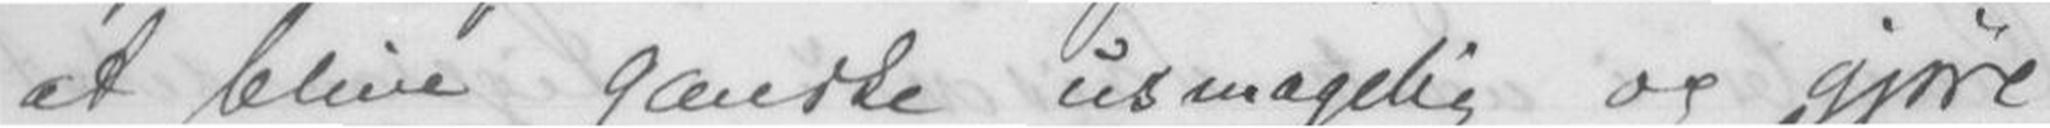at blive ganske usmagelig og gjøre
